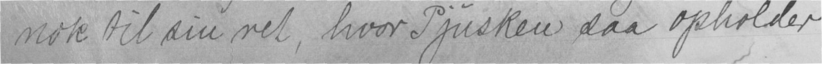nok til sin ret, hvor Pjusken saa opholder
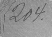204.
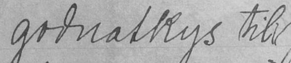godnatkys til
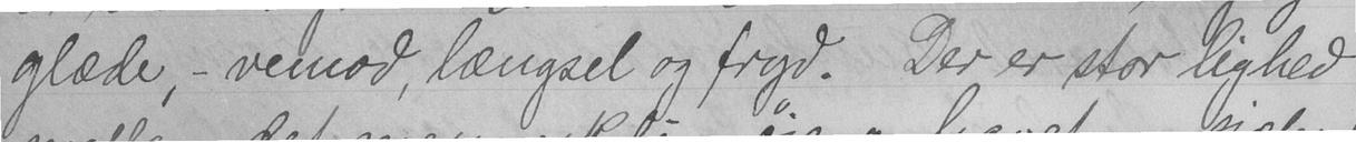glæde, - vemod, længsel og fryd. Der er stor lighed
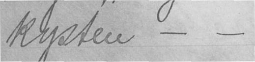kysten - -
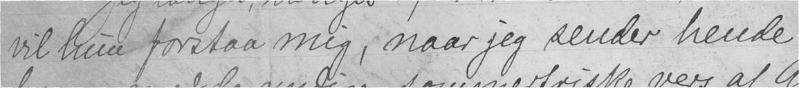vil hun forstaa mig, naar jeg sender hende
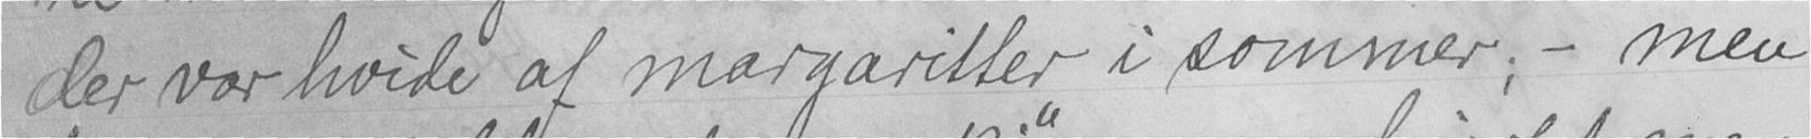der var hvide af margaritter i sommer; - men
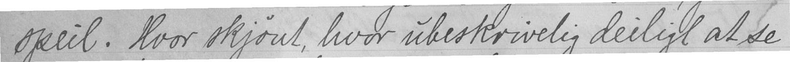speil. Hvor skjønt, hvor ubeskrivelig deiligt at se
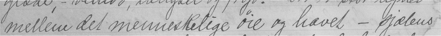mellem det menneskelige øie og havet - sjælens
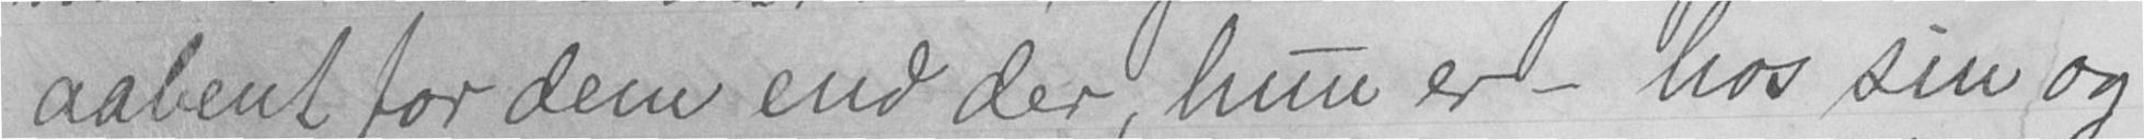aabent for dem end der, hun er - hos sin og
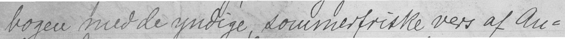bogen med de yndige, sommertriske vers af An-
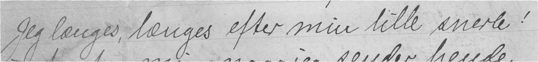Jeg længes, længes efter min lille snerle!
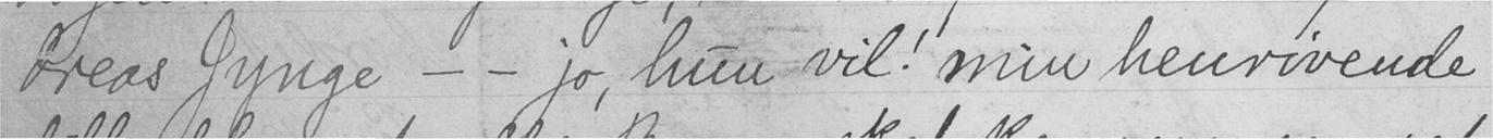dreas Jynge - - jo, hun vil! min henrivende
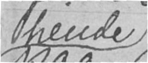hende
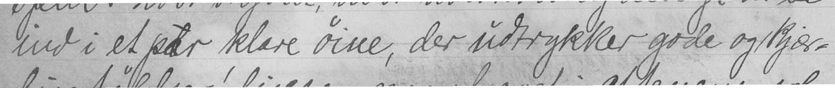ind i et par klare øine, der udtrykker gode og kjær-
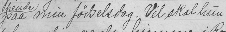paa min fødselsdag. Vel skal hun
